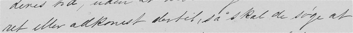ret eller adkomst dertil, så skal de søge at
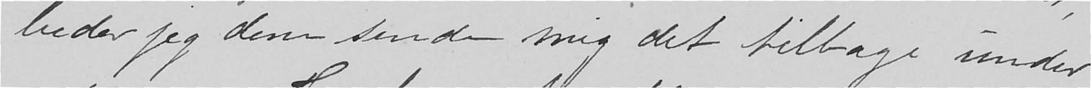beder jeg dem sende mig det tilbage under
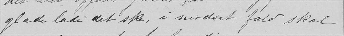glæde lade det ske, i modsat fald skal
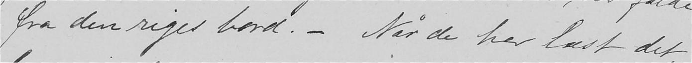fra den riges bord. - Når de har læst det
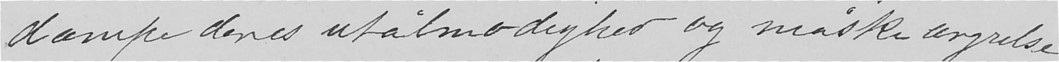dæmpe deres  utålmodighed og måske ærgrelse
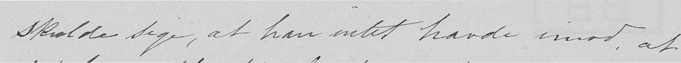skulde sige, at han intet havde imod at
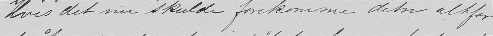Hvis det nu skulde forekomme dem altfor
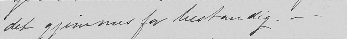det gjemmes for bestandig. - -
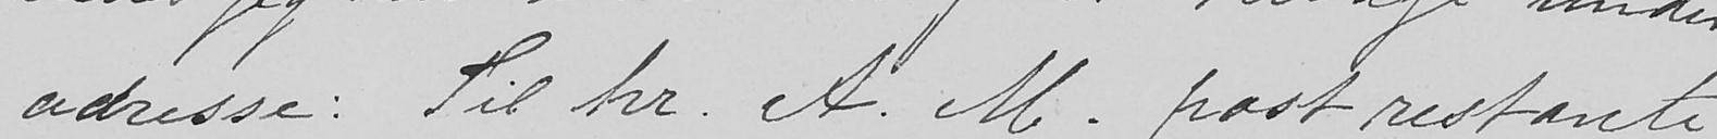adresse. Til hr. A. M. post restante
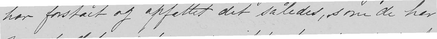har forstået og opfattet det således, som de har
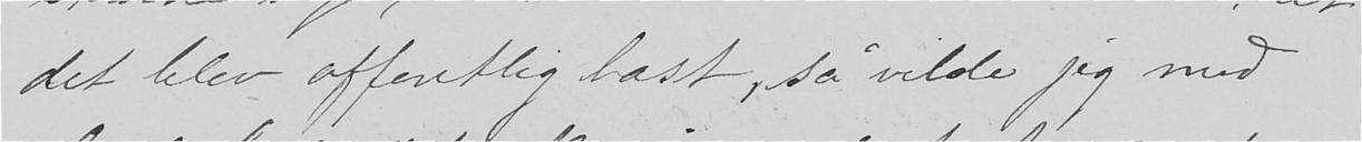det blev offentlig læst, så vilde jeg med
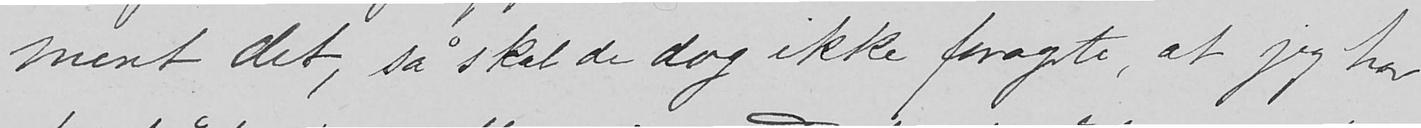ment det, så skal de dog ikke foragte, at jeg har
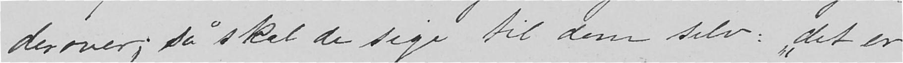derover; så skal de sige til dem selv: "det er
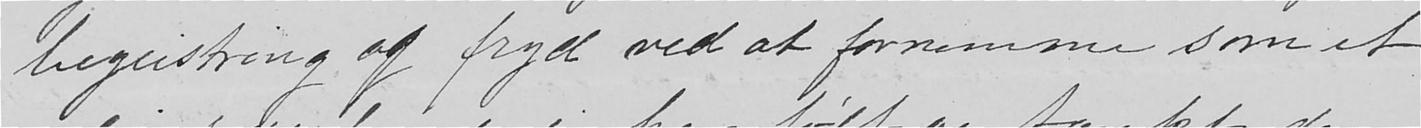begeistring og fryd ved at fornemme som et
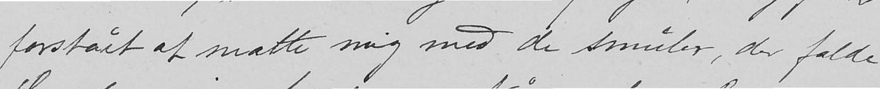forstået at mætte mig med de smuler, der falde
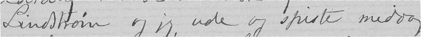Lindstrøm og jeg ude og spiste middag
